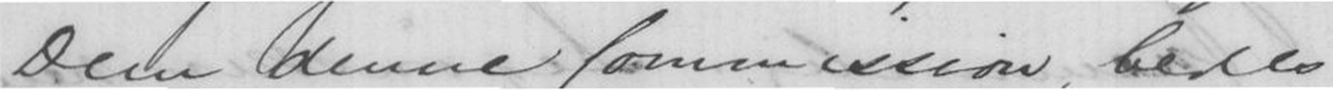Dem denne Commission, bedes
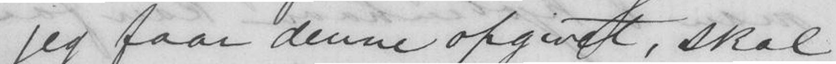jeg faar denne opgivet, skal
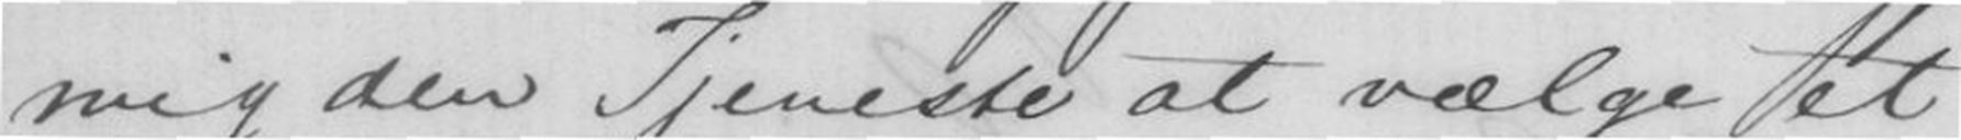mig den Tjeneste at vælge et
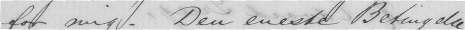for mig. Den eneste Betingelse
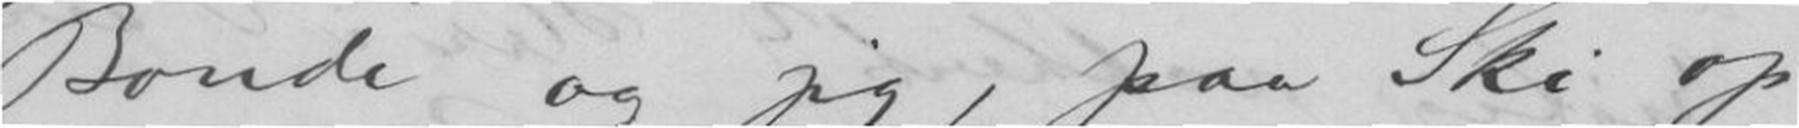Bonde og jeg, paa Ski op
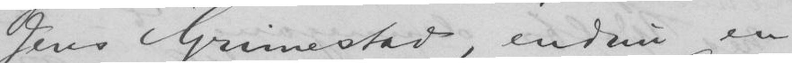Jens Grimstad, endnu en
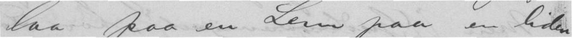laa paa en Lem paa en liden
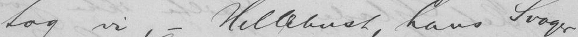tog vi, - Hellebust, hans Svoger
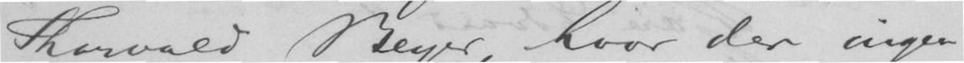Thorvald Beyer, hvor der ingen
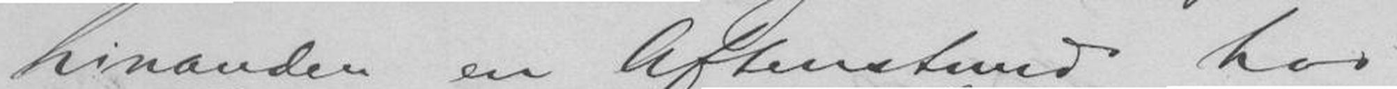hinanden en Aftenstund hos
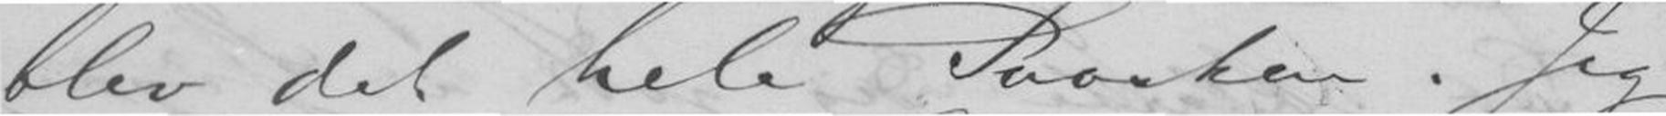blev det hele Paasken. Jeg
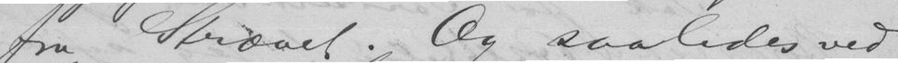fra Strævet. Og saaledes ved-
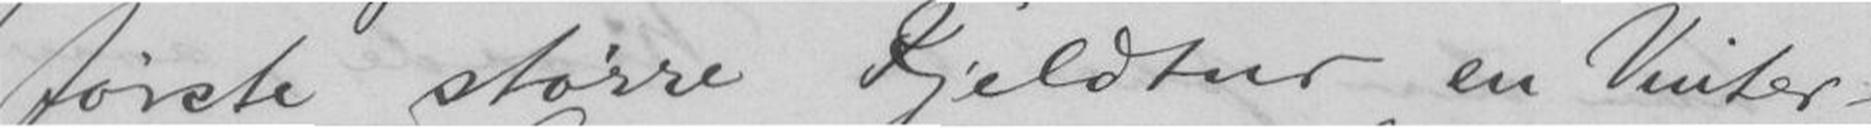første større Fjeldtur en Vinter-
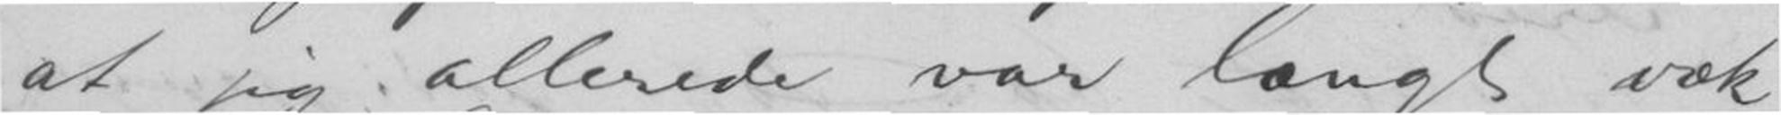at jeg allerede var langt væk
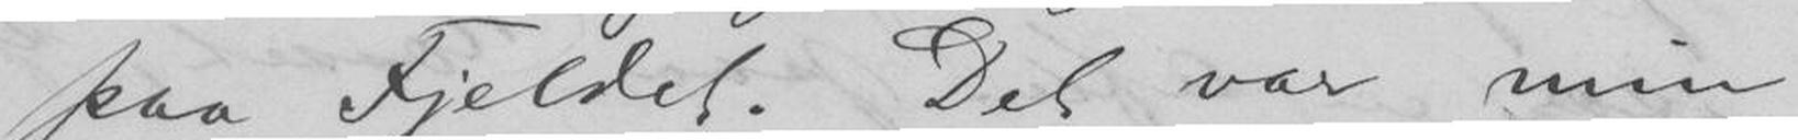paa Fjeldet. Det var min
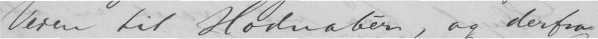Veien til Hodnabér, og derfra
